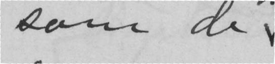som de
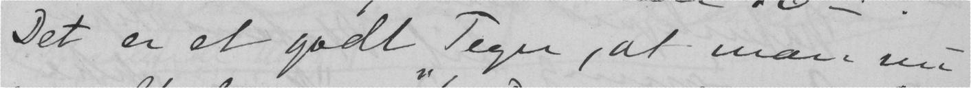Det er et godt Tegn, at man nu
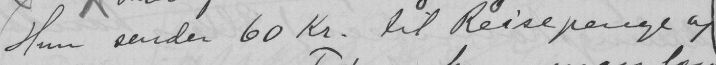Hun sender 60 kr. til Reisepenge og
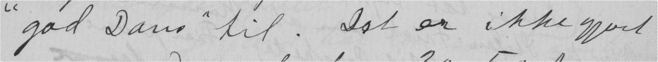"god Dans" til. Det er ikke gjort
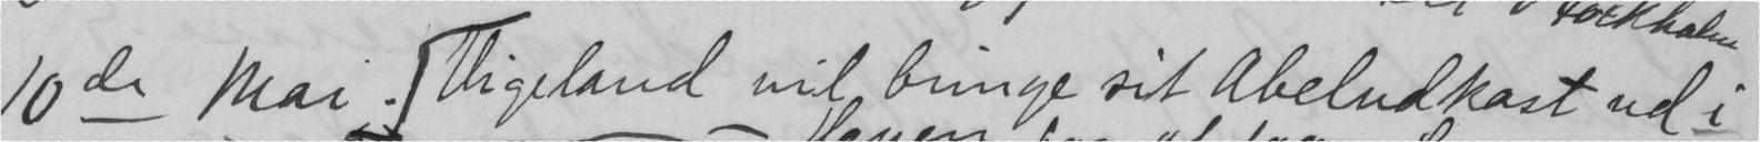10de mai. Vigeland vil bringe sit Abeludkast ud i
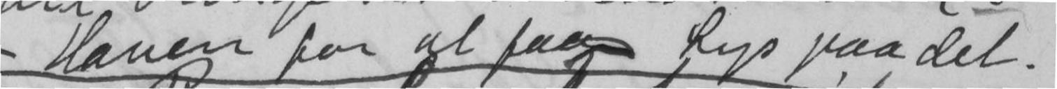Haven for at faa Lys paa det.
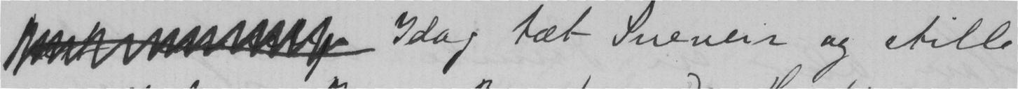Idag tæt Sneveir og stille
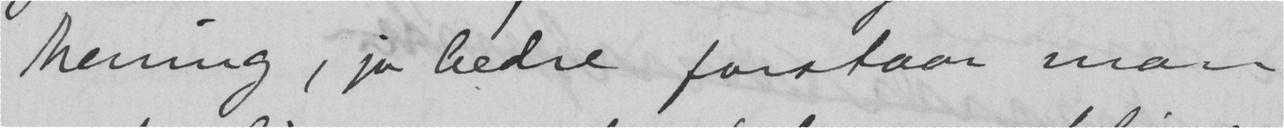Mening, jo bedre forstaar man
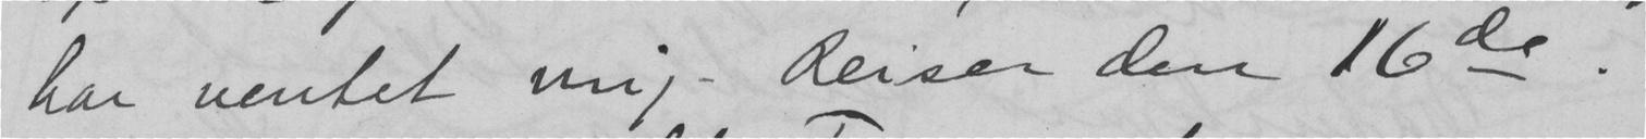har ventet mig. Reiser den 16de.
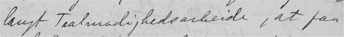langt Taalmodighedsarbeide, at faa
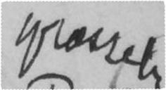græsselig
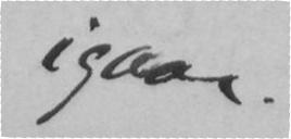igaar.
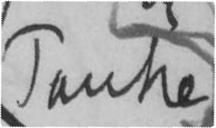Tanke
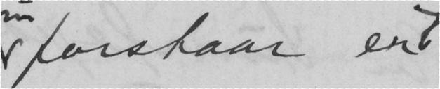forstaar er
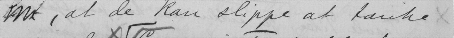, at de kan slippe at tænke
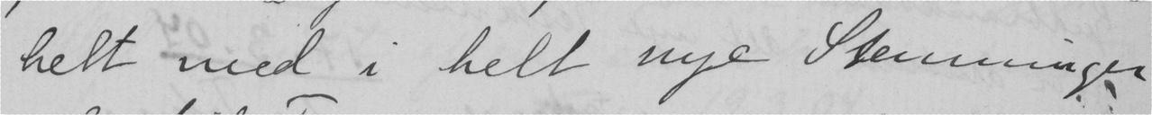helt med i helt nye Stemninger
